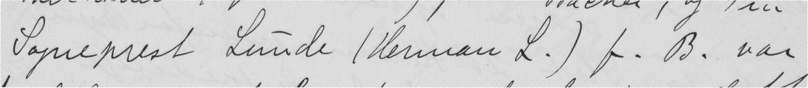Sogeprest Lunde (Herman L.) f. B. var
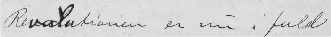Revolutionen er nu i fuld
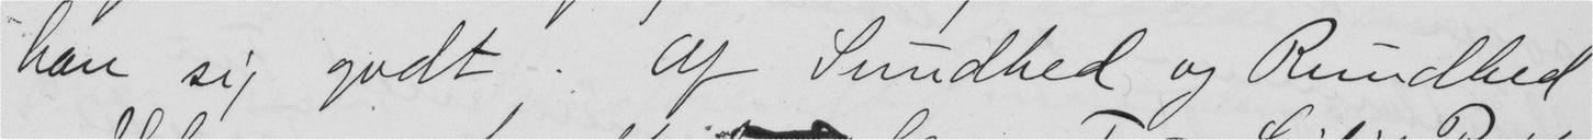han sig godt. Af Sundhed og Rundhed
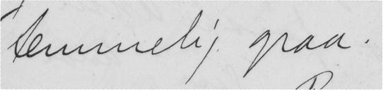temmelig graa.
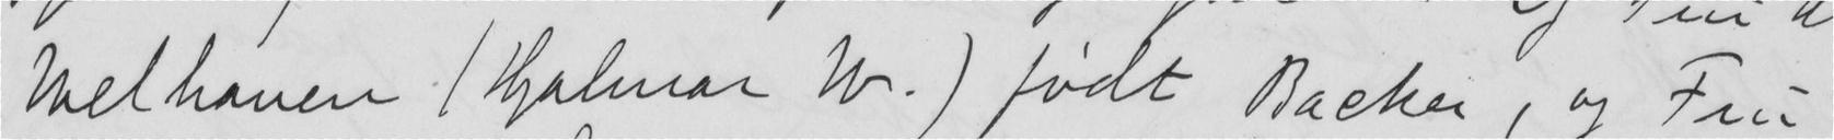Welhaven (Hjalmar W.) født Backer, og Fru
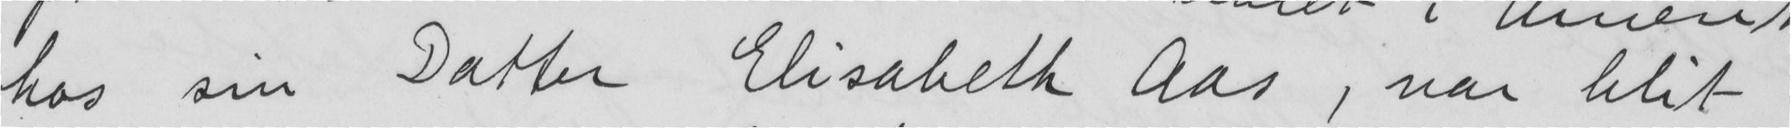hos sin Datter Elisabeth Aas, var blit
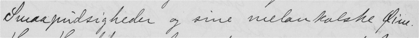Smaapudsigheder og sine melankolske Øine.
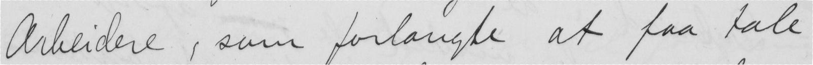Arbeidere, som forlangte at faa tale
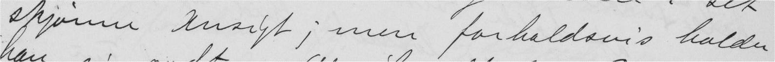skjønne Ansigt; men forholdsvis holder
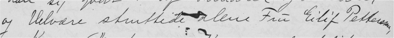og Velvære struttede alene Fru Eilif Pettersen
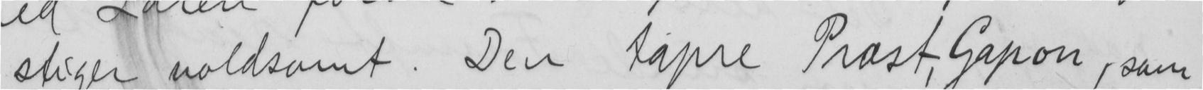stiger voldsomt. Den tapre Præst, Gapon, som
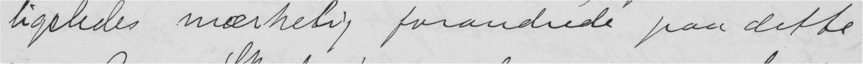ligeledes mærkelig forandrede paa dette
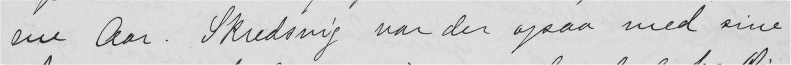ene Aar. Skredsvig var der ogsaa med sine
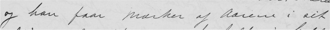og han faar Mærker af Aarene i sit
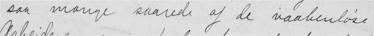saa mange saarede af de vaabenløse
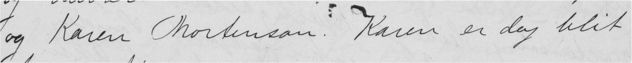og Karen Mortenson. Karen er dog blit
